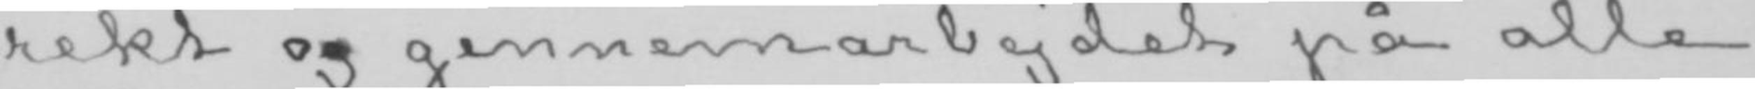rekt og gennemarbejdet på alle
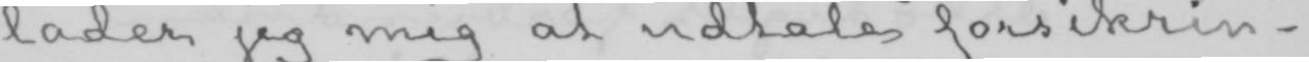lader jeg mig at udtale forsikrin-
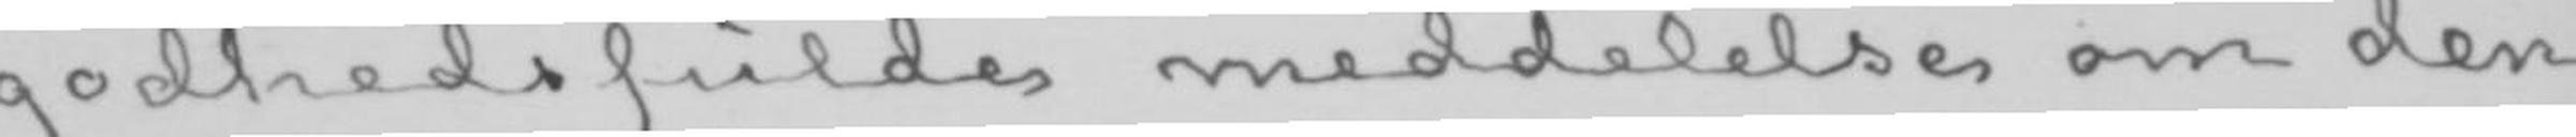godhedsfulde meddelelse om den
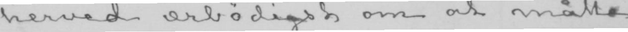herved ærbødigst om at måtte
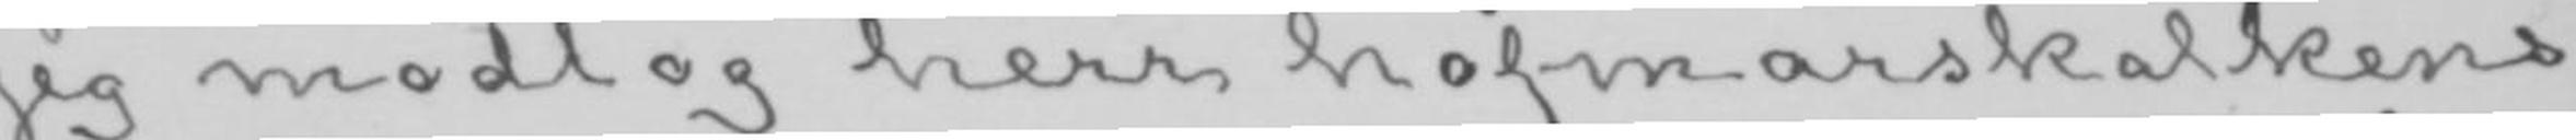jeg modtog herr hofmarskalkens
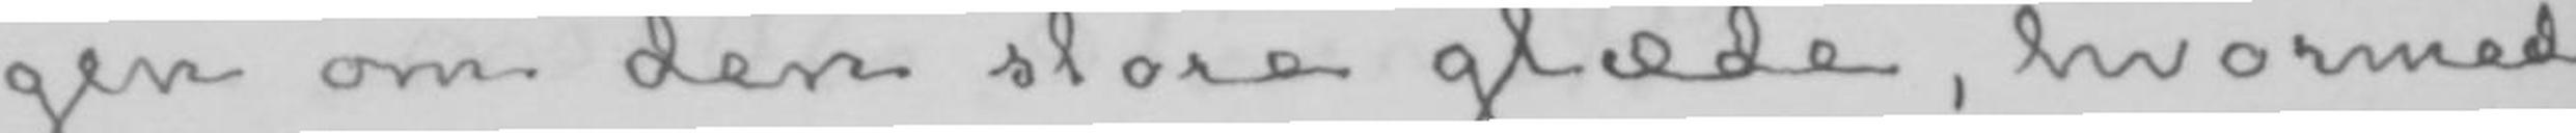gen om den store glæde, hvormed
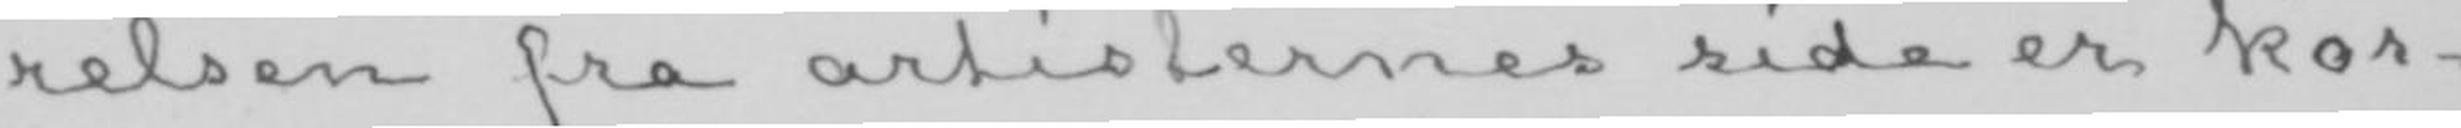relsen fra artisternes side er kor-
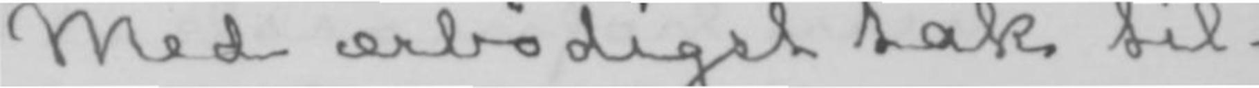Med ærbødigst tak til-
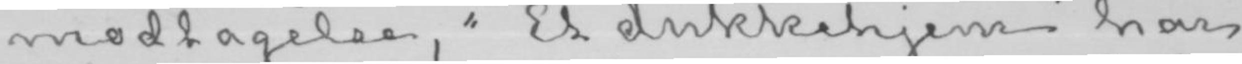modtagelse, «Et dukkehjem» har
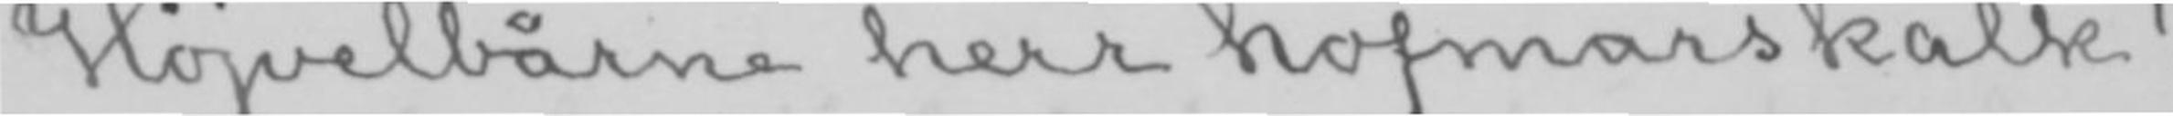Højvelbårne herr hofmarskalk!
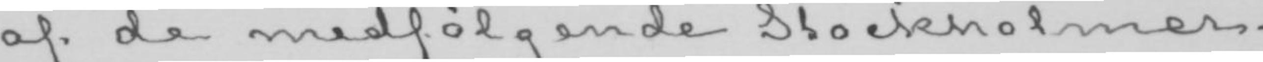af de medfølgende Stockholmer-
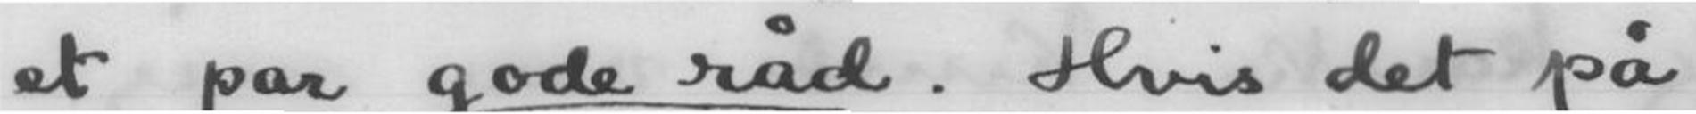et par gode råd. Hvis det på
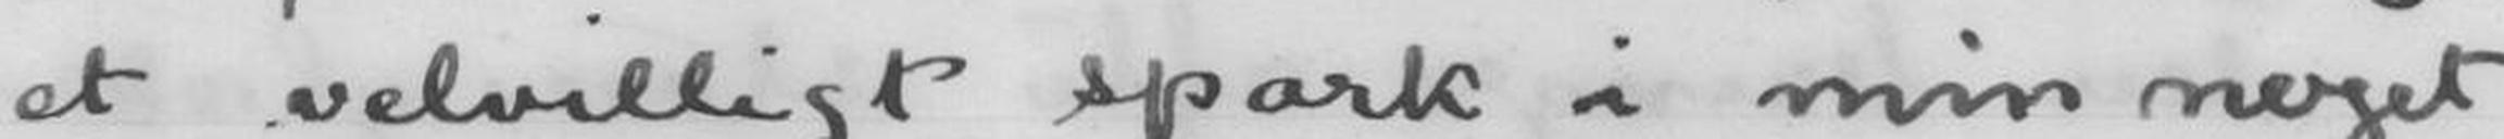et velvilligt spark i min noget
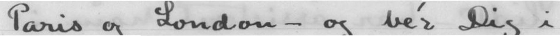Paris og London - og be'r Dig i
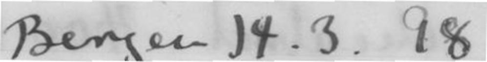Bergen 14.3.98
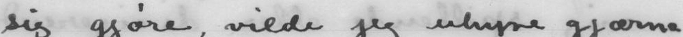sig gjøre, vilde jeg uhyre gjærne
